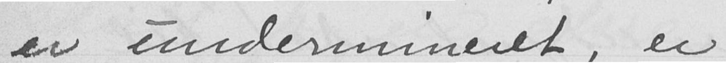er undermineret, er
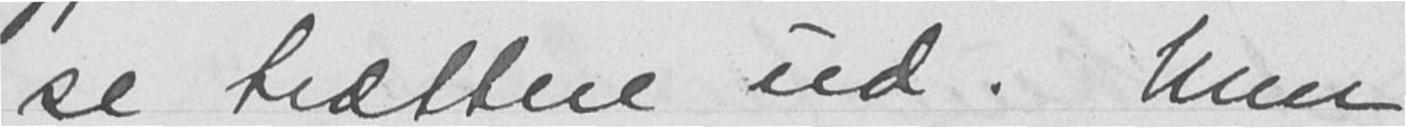se trættere ud. Men
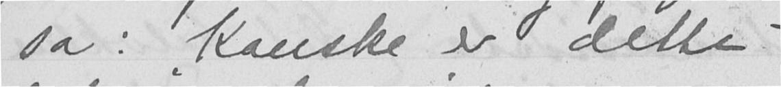sa: "Kanske er dette
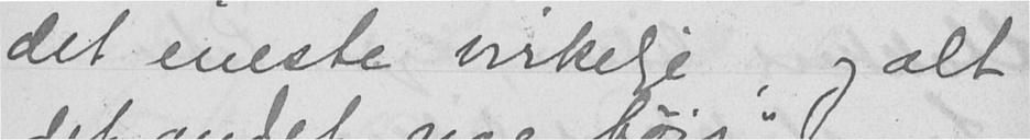det eneste virkelige, og alt
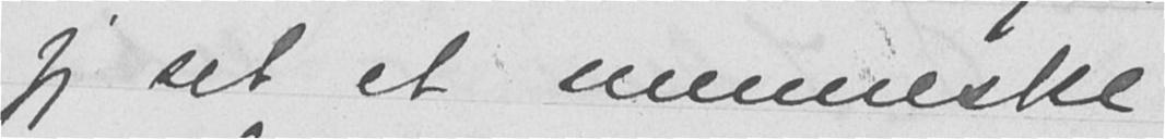jeg set et menneske
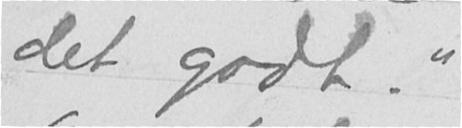det godt."
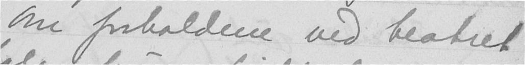Om forholdene ved teatret
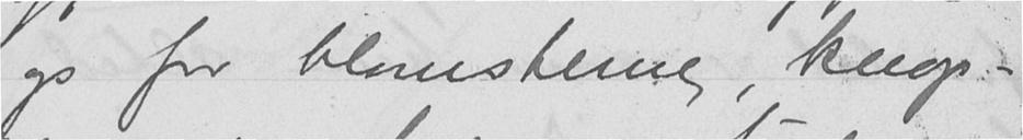op for blomsterne, knop-
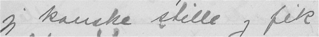jeg kanske stille og fik
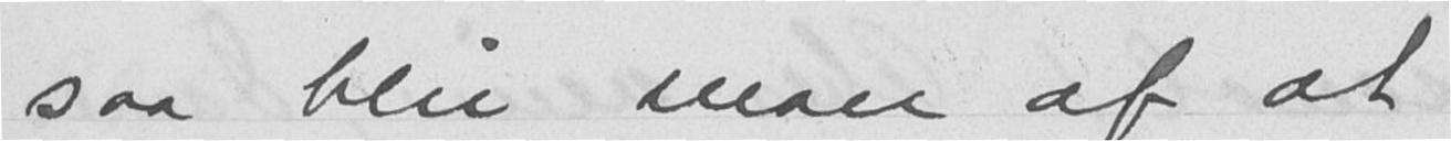saa blir man af at
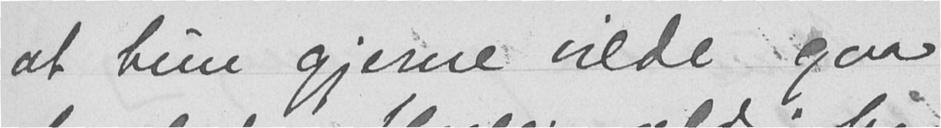at hun gjerne vilde gaa
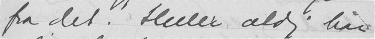fra det. Heller aldrig har
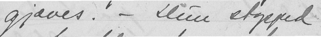gjæves. - Hun stopped
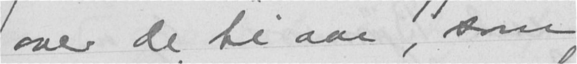over de ti aar, som
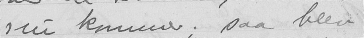nu kommer; saa blev
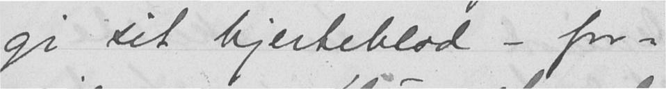gi sit hjerteblod - for-
